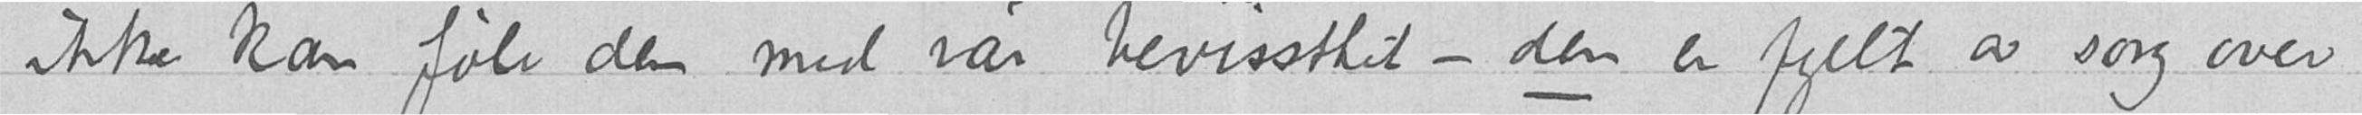ikke kan føle den med vår bevissthet – den er fyllt av sorg over
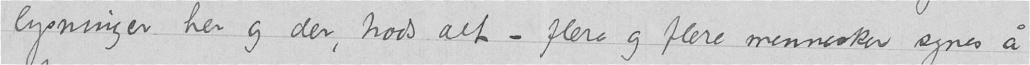lysninger her og der, trods alt – flere og flere mennesker synes å
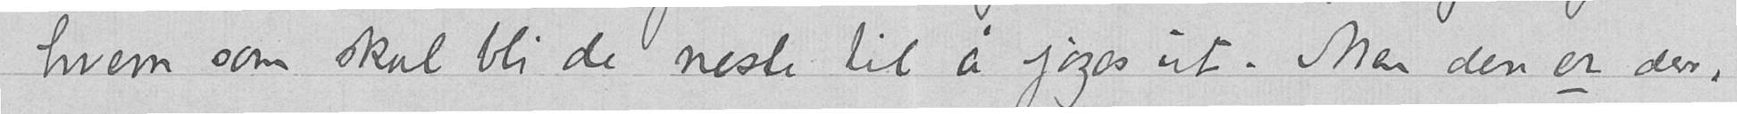hvem som skal bli de neste til å jages ut. Men den er der,
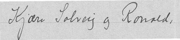Kjære Solveig og Ronald,
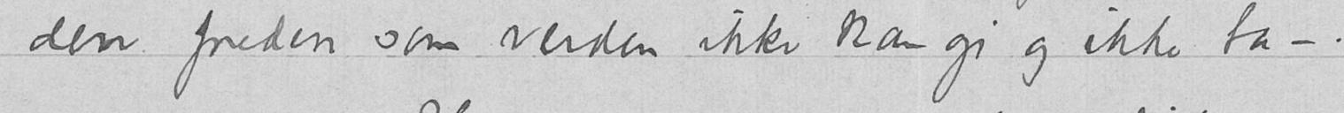den freden som verden ikke kan gi og ikke ta –.
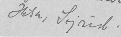Hilsen, Sigrid.
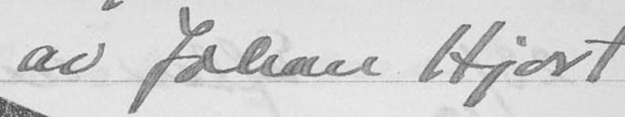av Johan Hjort
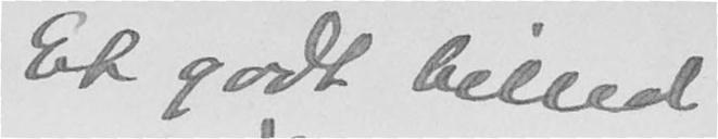Et godt billed
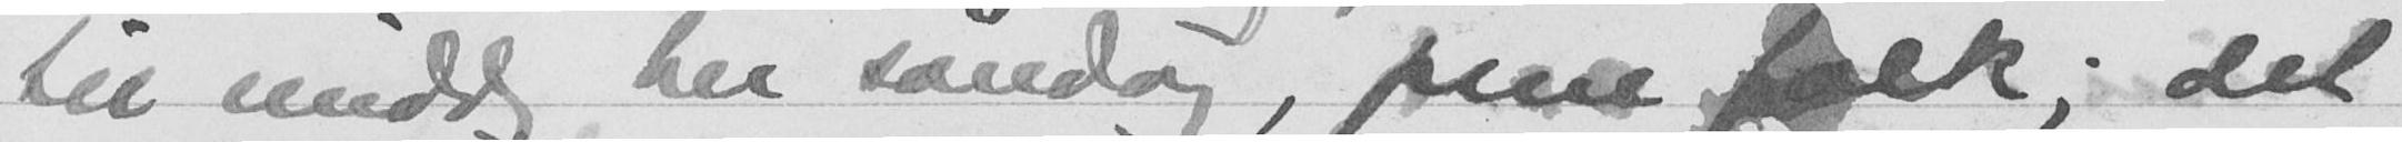til middag her søndag, pene folk; det
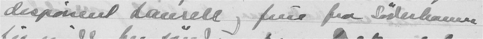disponent Lansell og frue fra Søderhavn
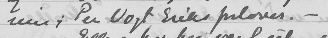inn i Per Vogt Eriks forlover. -
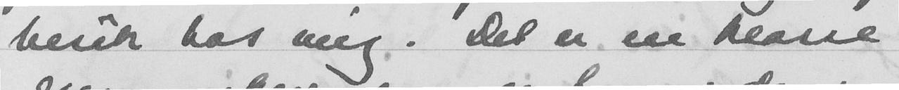besøk hos mig. Det er en klasse
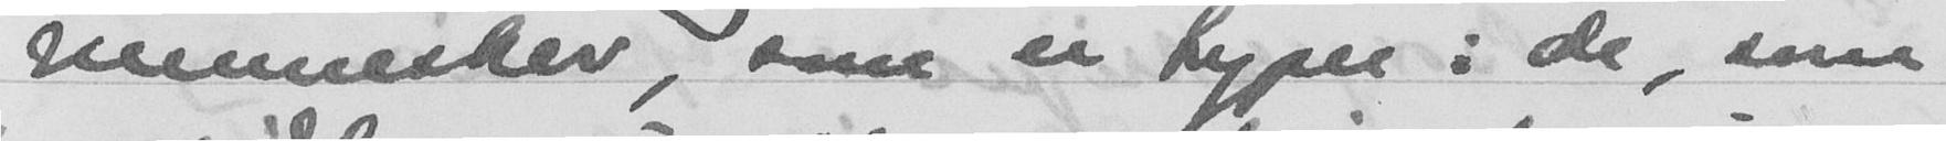mennesker, som er typer: de, som
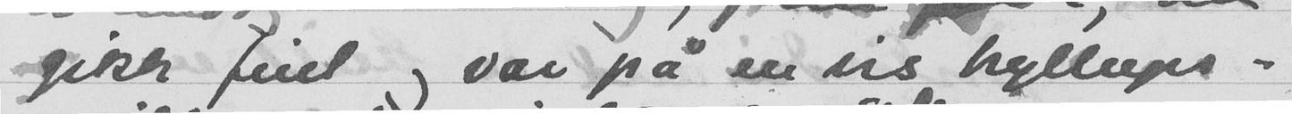gikk fint og var på en vis bryllups-
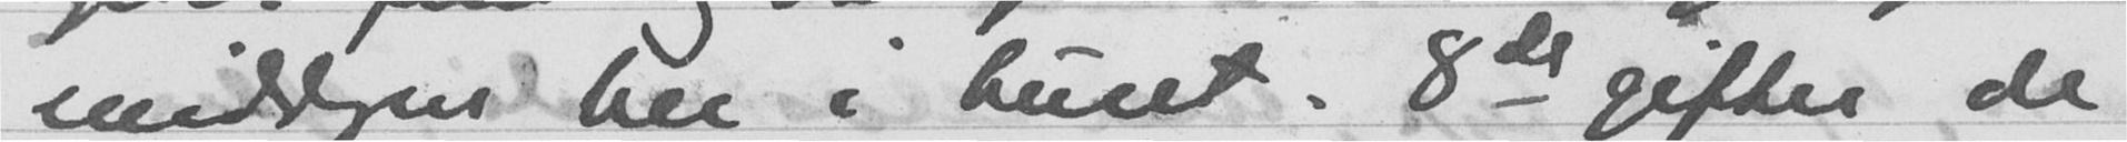middagen her i huset. 8de gifter de
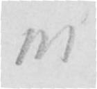191
